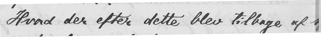Hvad der efter dette blev tilbage af sym-
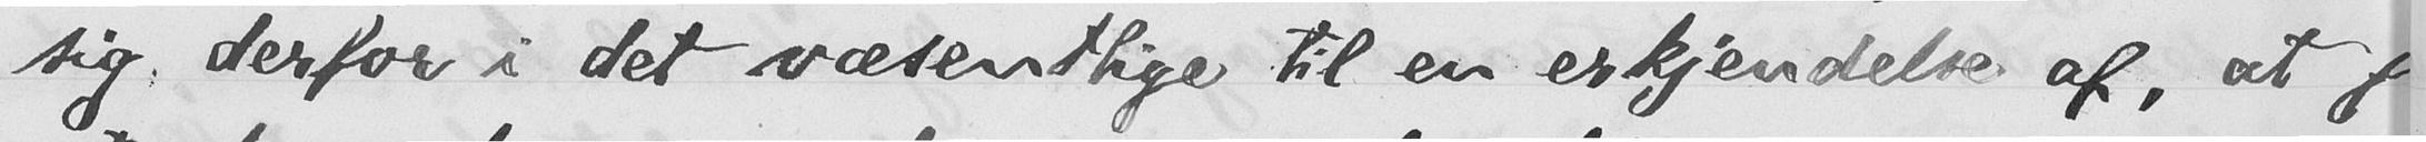sig derfor i det væsentlige til en erkjendelse af, at
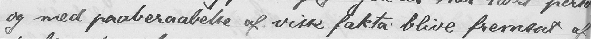og med paaberaabelse af visse fakta blive fremsat af
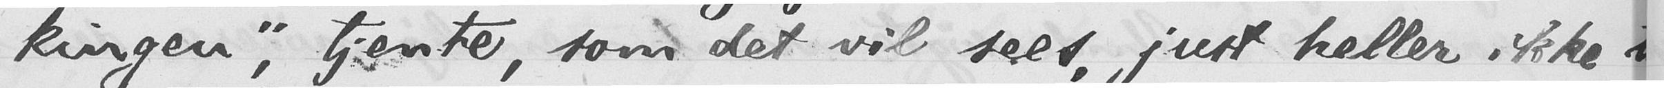kingen", tjente, som det vil sees, just heller ikke
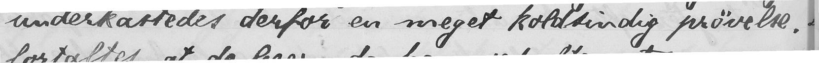underkastedes derfor en meget koldsindig prøvelse.
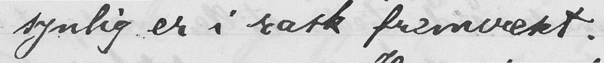synlig er i rask fremvæxt.
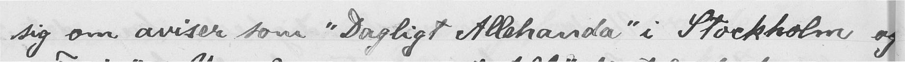sig om aviser som "Dagligt Allehanda" i Stockholm og
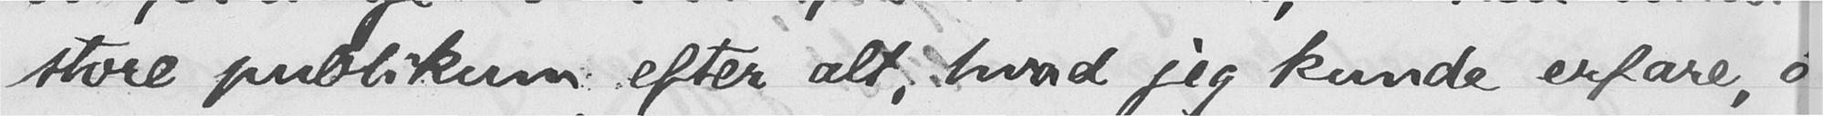store publikum efter alt, hvad jeg kunde erfare, o
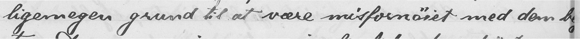ligemegen grund til at være misfornøiet med dem b
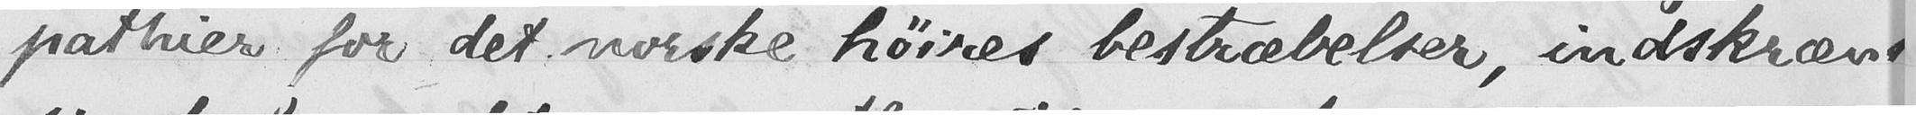pathier for det norske høires bestræbelser, indskræn
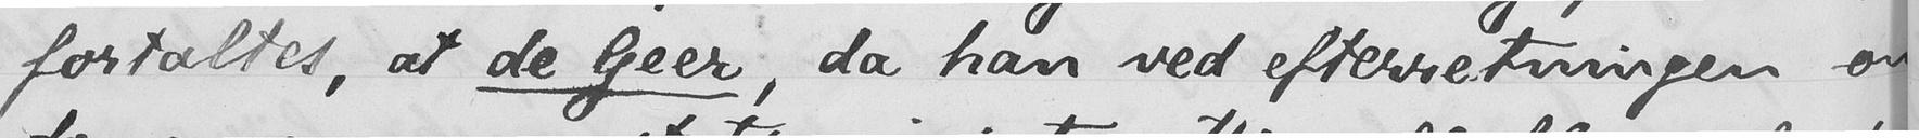fortaltes, at de Geer, da han ved efterretningen om
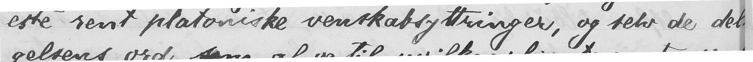este rent platoniske venskabsyttringer, og selv de de
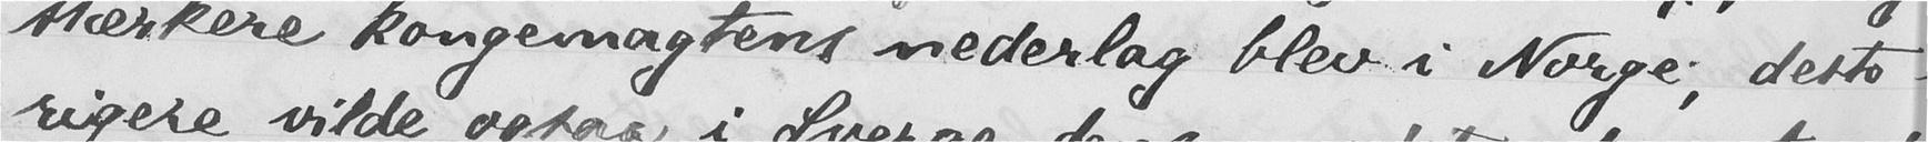stærkere kongemagtens nederlag blev i Norge, desto
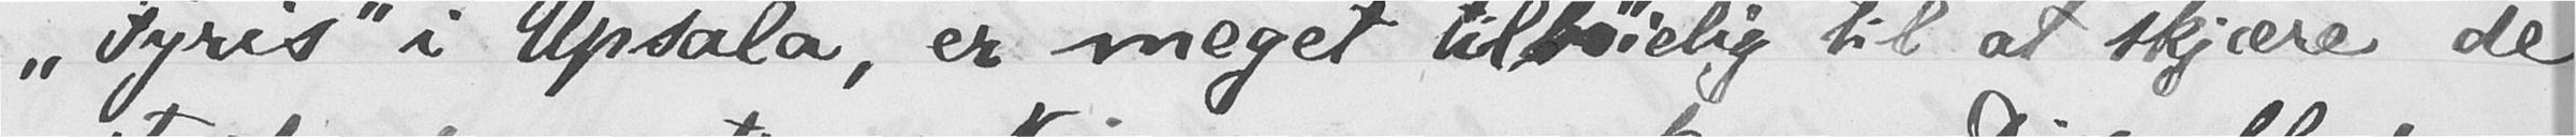"Fyris" i Upsala, er meget tilbøielig til at skjære de
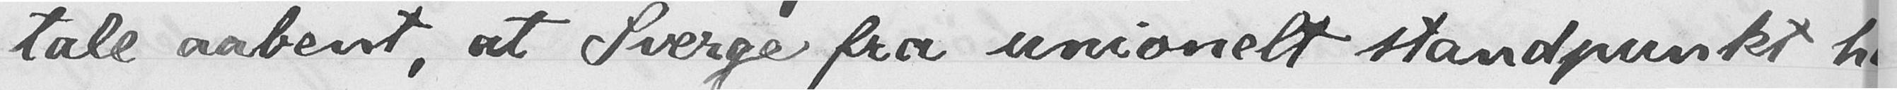tale aabent, at Sverge fra unionelt standpunkt
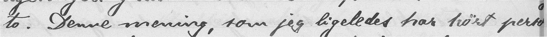to. Denne mening, som jeg ligeledes har hørt pers
